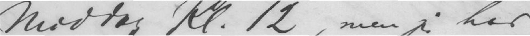Middag Kl. 12, men jeg har
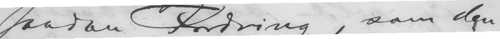saadan Fordring, som den
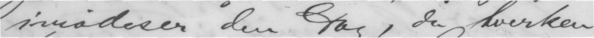imødeser den Dag, da hverken
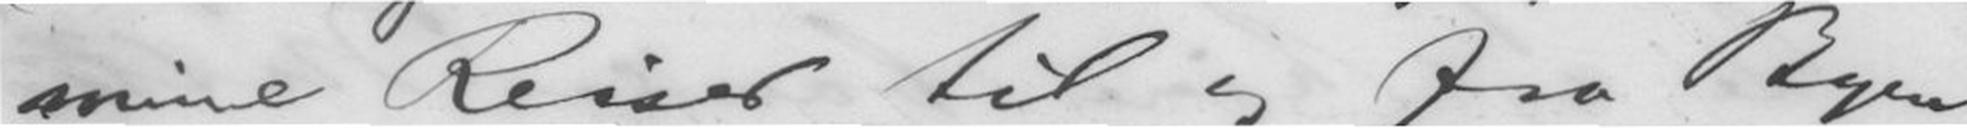mine Reiser til og fra Byen
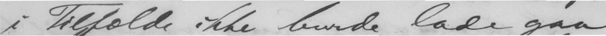i Tilfælde ikke burde lade gaa
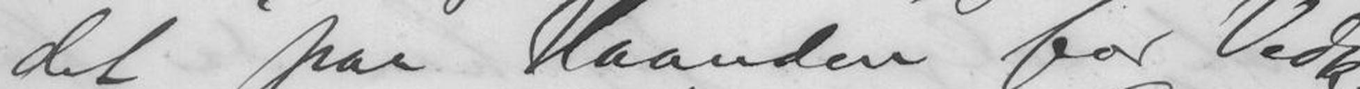det paa Haanden for Vedk.
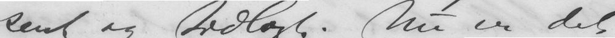sent og tidligt. Nu er det
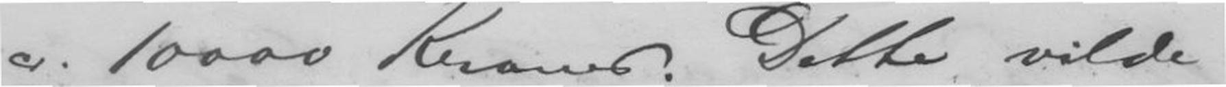ca. 10000 Kroner. Dette vilde
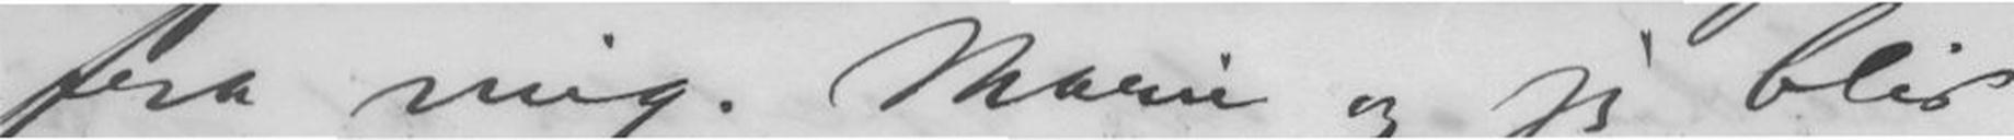fra mig. Marie og jeg blir
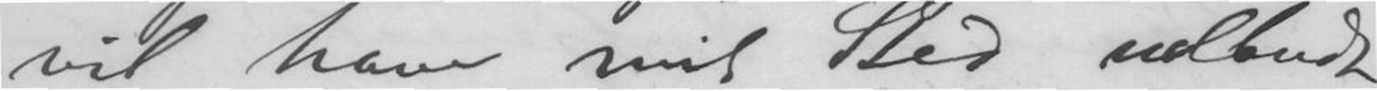vil have mit Sted udbudt
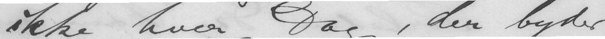ikke hver Dag, der byder
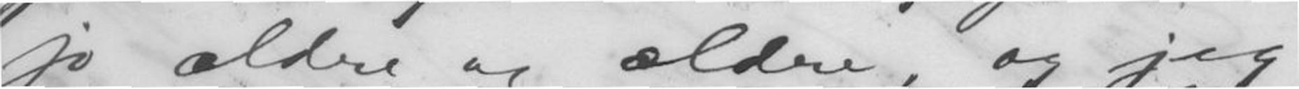jo ældre og ældre, og jeg
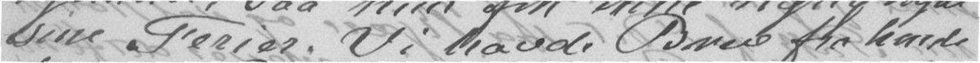sine Ferier. Vi havde Brev fra hende
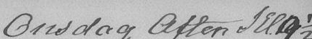Onsdag Aften Kl 9½
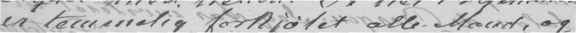er temmelig forkjølet alle Mand, og
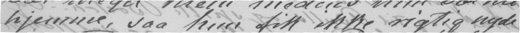hjemme, saa hun fik ikke rigtig nyde
